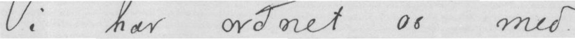Vi har ordnet os med
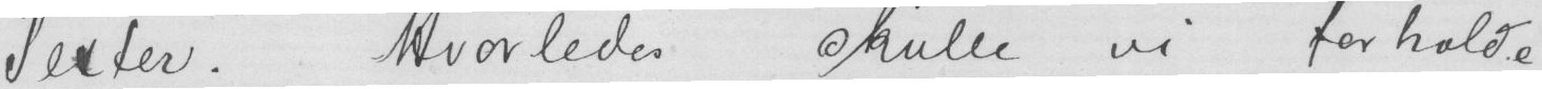Texter. Hvorledes skulle vi forholde
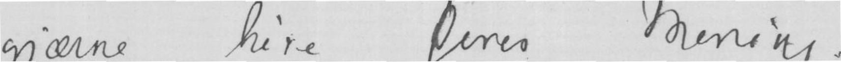gjærne høre Deres Mening.-
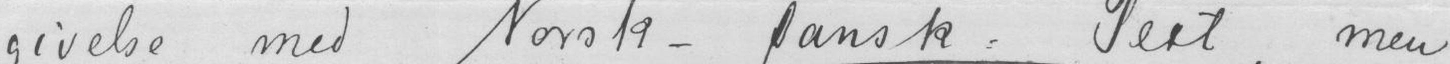givelse med Norsk - Dansk Text, men
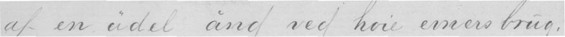af en ädel ånd ved høie evners brug.
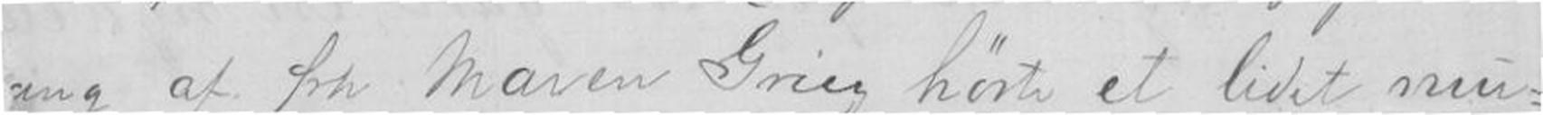ing af frk Maren Grieg hørte et lidet mu-
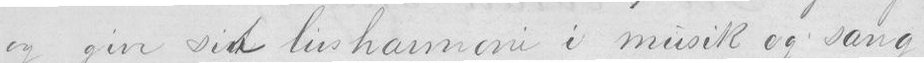og give sit sinlivsharmoni i musik og sang
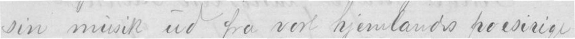sin musik ud fra vort hjemlands poesirige
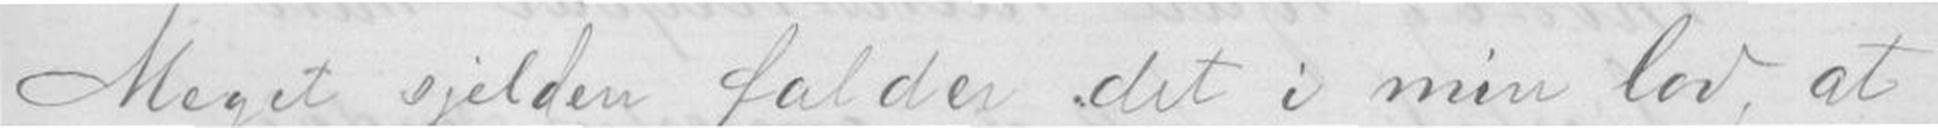Meget sjelden falder det i min lod, at
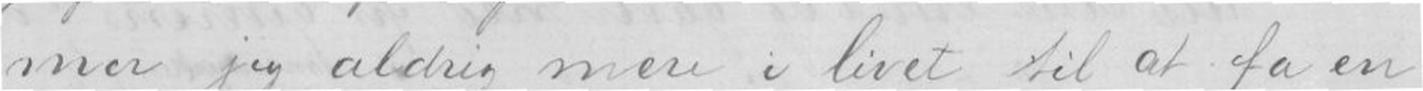mer jeg aldrig mere i livet til at få en
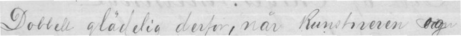Dobbelt glädelig derfor, når kunstneren og
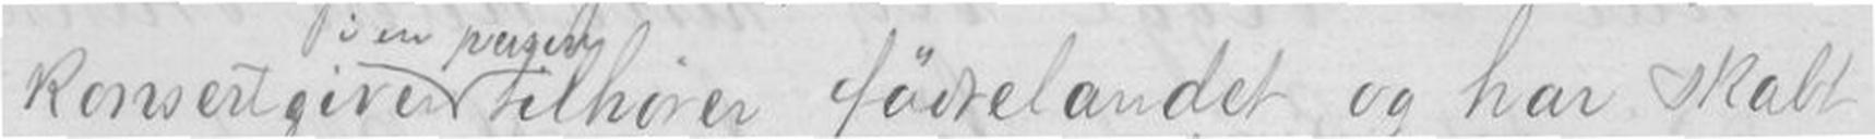konsertgiver tilhører fädrelandet og har skabt
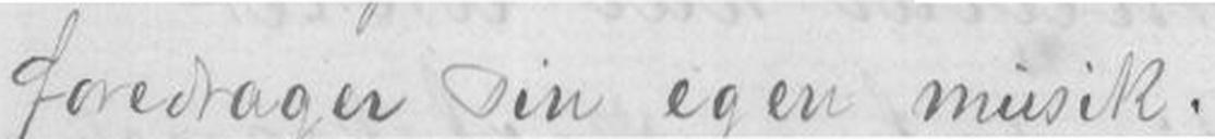foredrager sin egen musik.
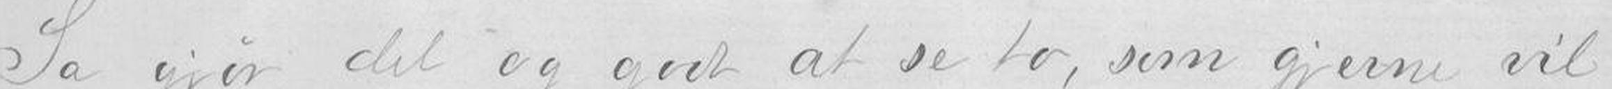Så gjør det og godt at se to, som gjerne vil
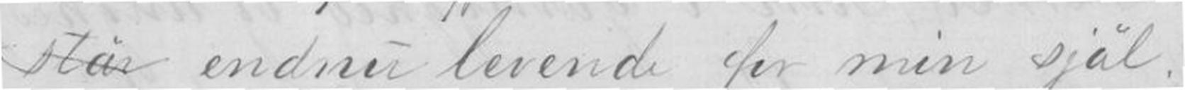står endnu levende for min själ.
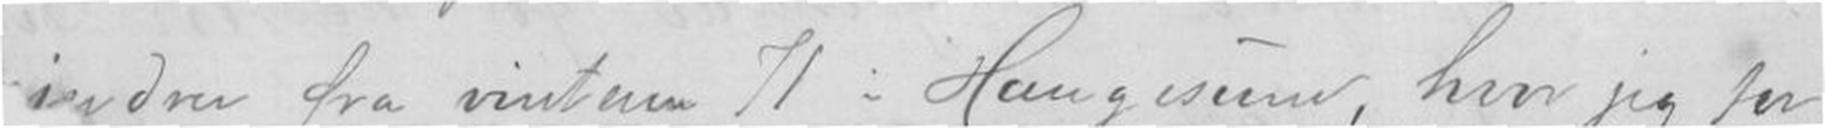den fra vinteren 71 i Haugesund, hvor jeg for
